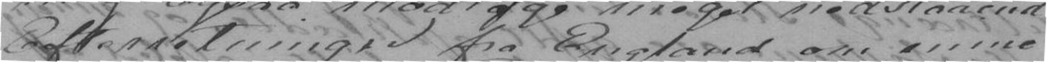Efterretninger fra England om mine
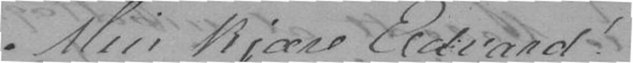Min kjære Edvard!
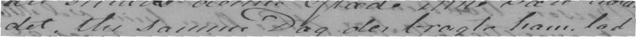det, thi samme Dag der bragte ham, lod
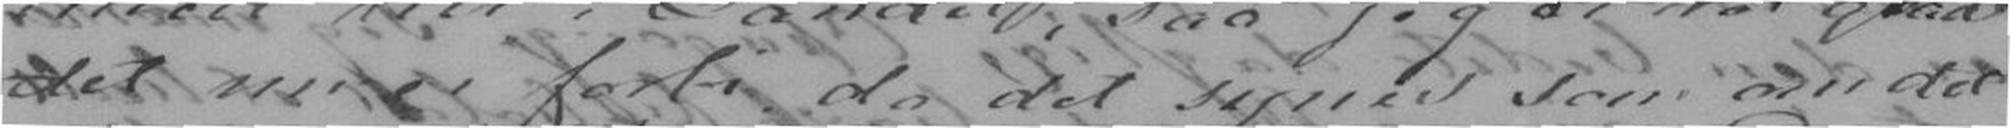det nu er forbi da det synes som om det
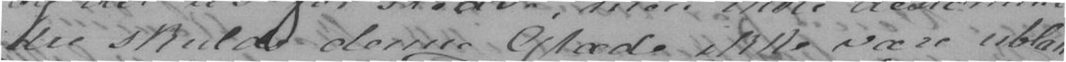dre skulde denne Glæde ikke være ublan-
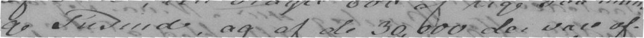ge Tusinde, og de 30.000 der vare af-
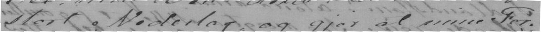stort Nederlag og gjør at mine For-
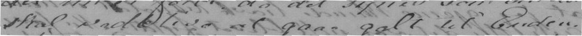skal vedblive at gaae galt til Enden.
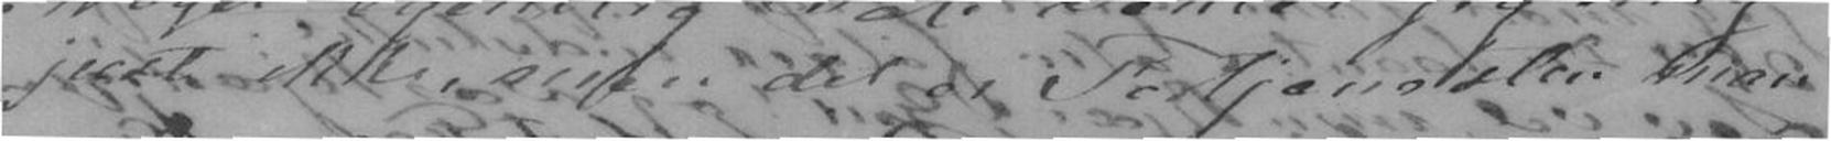just ikke, men det er Fortjenesten man
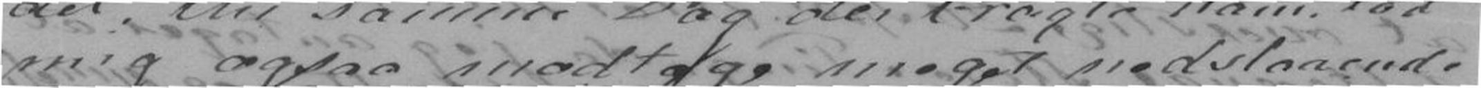mig ogsaa modtage meget nedslaaende
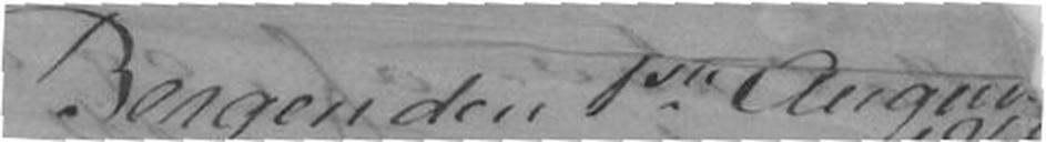Bergen den 1ste August
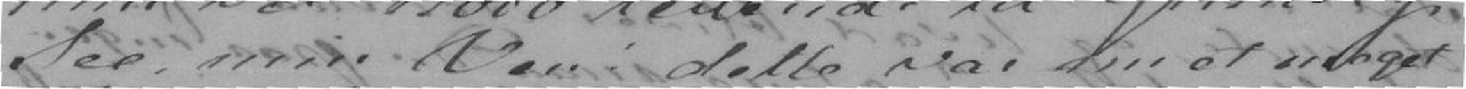See min Ven! dette var nu et meget
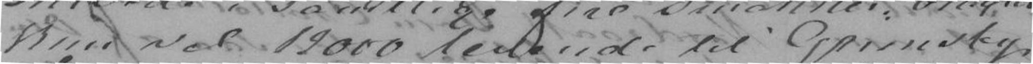kun vel 12.000 levende til Grimsby.
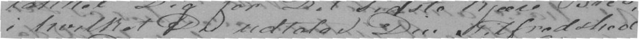i hvilket Du udtaler Din Tilfredshed
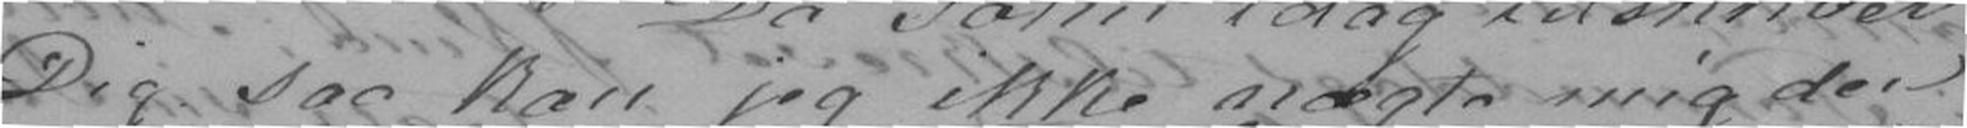Dig saa kan jeg ikke nægte mig den
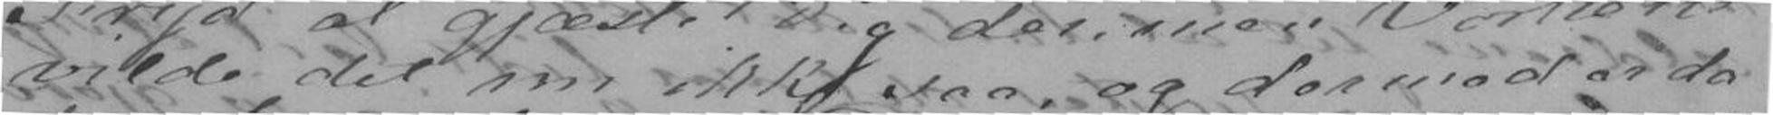vilde det nu ikke saa, og dermed er da
